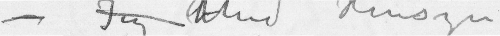– Jeg Med Hensyn
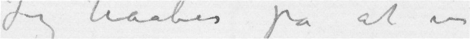Jeg haaber på at en
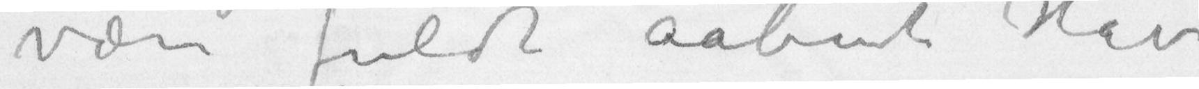være fuldt aabent Hav
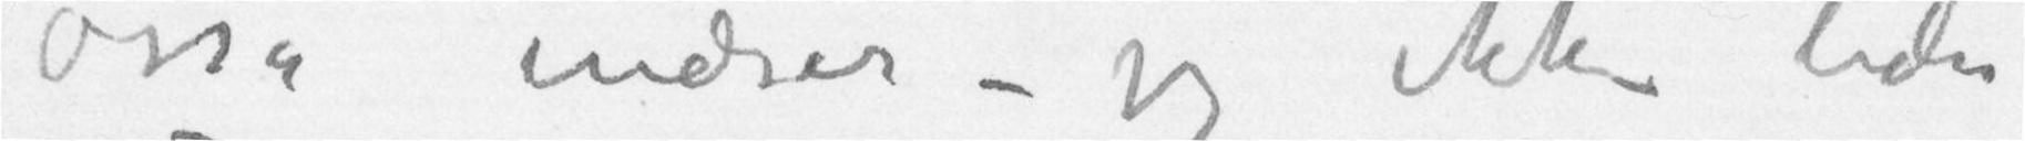osså indser – jeg ikke lider
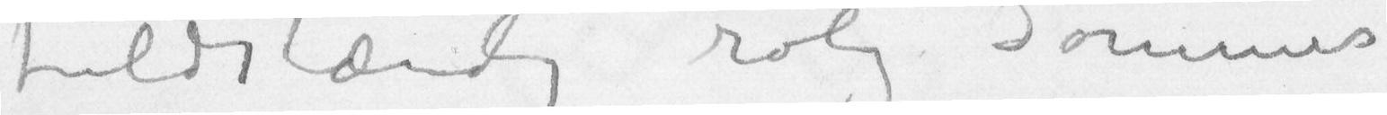fuldstændig rolig Sommer
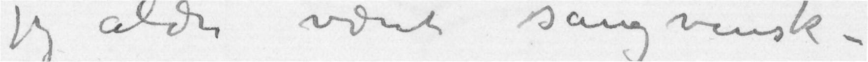jeg aldri været sangvinsk –
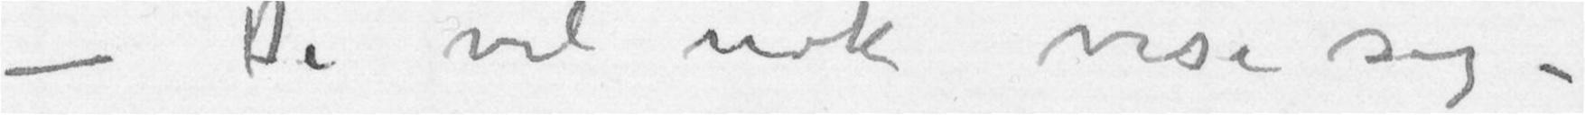– Det vil nok vise sig –
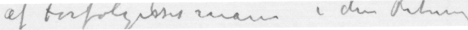af Forfølgelsesmani i den Retning
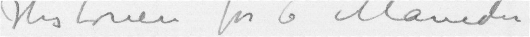Historien for 6 Måneder
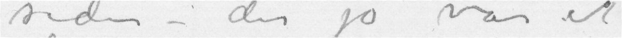siden – der jo var et
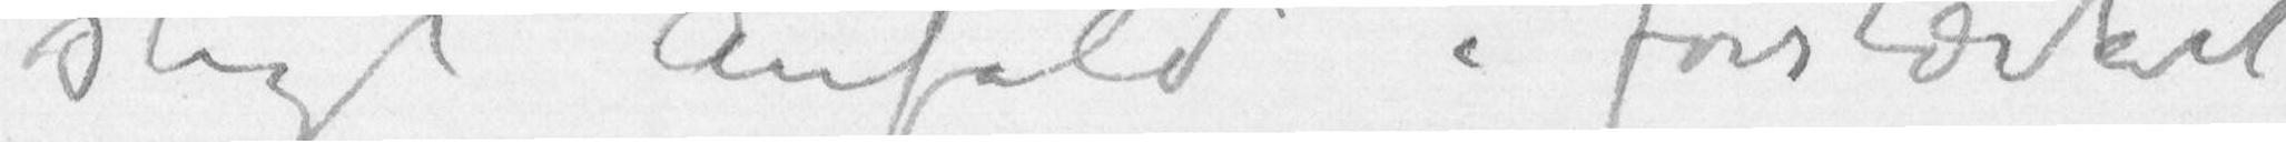sligt Anfald i forstærket
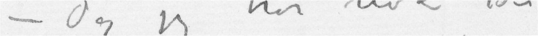– Og jeg tror nok Du
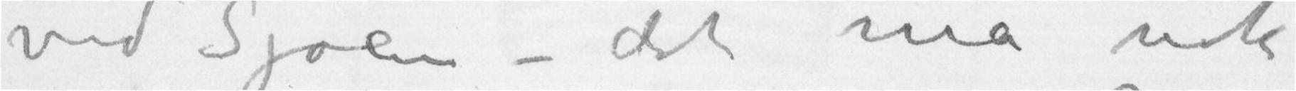ved Sjøen – det må nok
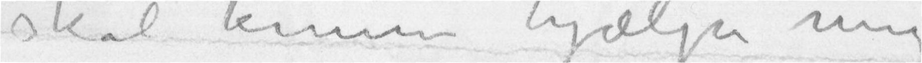skal kunne hjælpe mig
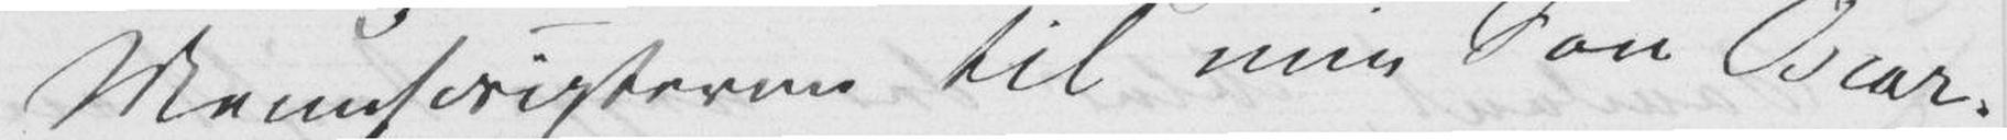Manuscripterne til min Søn Oscar.
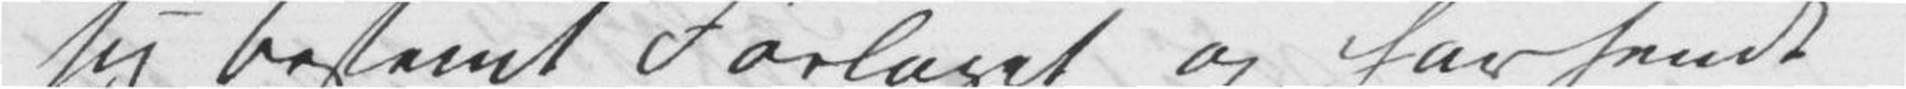sig bestemt Forlaget og har sendt
